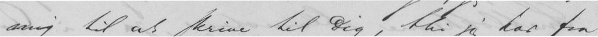mig til at skrive til Dig, thi jeg har fra
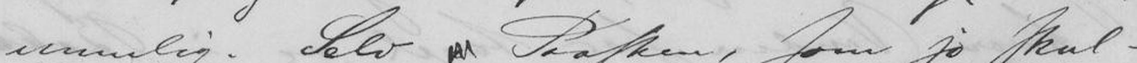umulig. Selv Paasken, som jo skul-
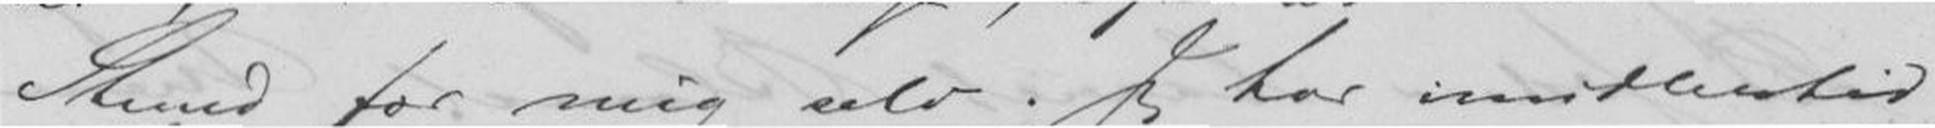Stund for mig selv. Jeg har imidlertid
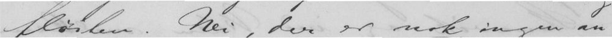fløiten. Nei, der er nok ingen an-
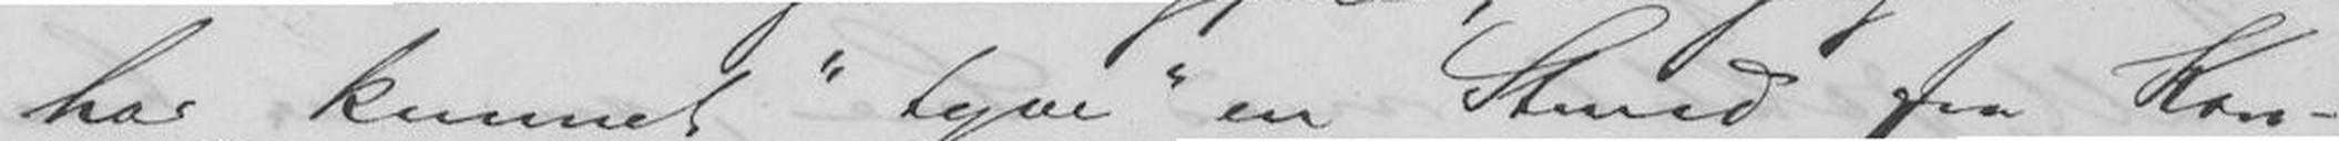har kunnet "tyve" en Stund fra Kon-
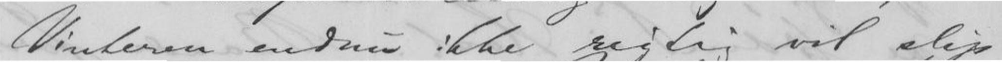Vinteren endnu ikke rigtig vil slip-
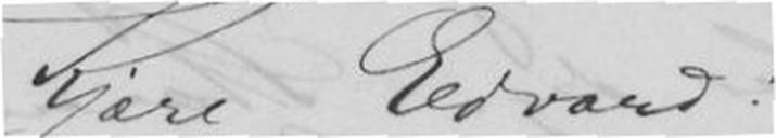Kjære Edvard!
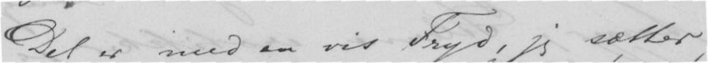Det er med en vis Fryd, jeg sætter
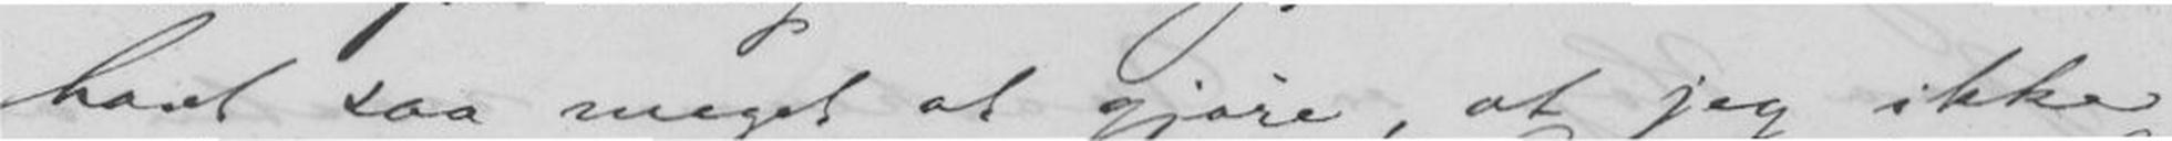havt saa meget at gjøre, at jeg ikke
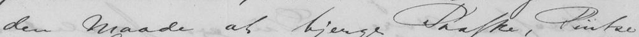den Maade at bjerge Paaske, Pintse
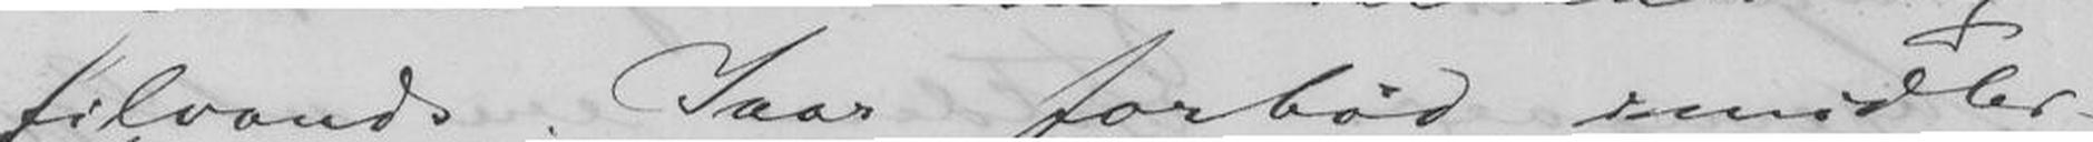tilvands. Iaar forbød imidler-
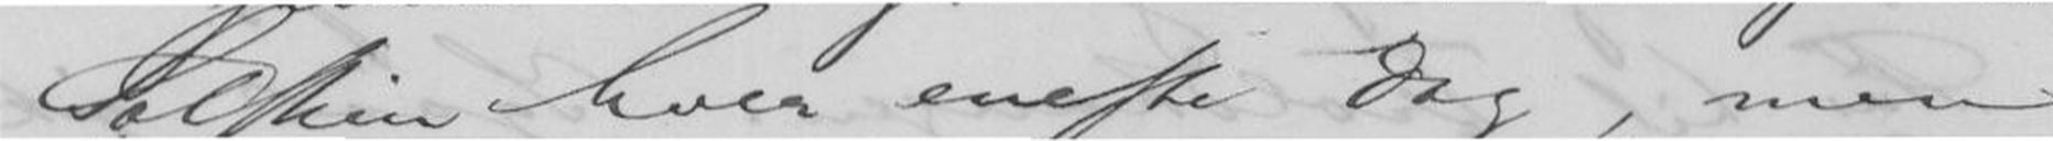Solskin hver eneste Dag, men
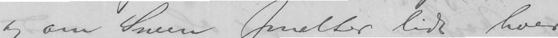og om Sneen smelter lidt hver
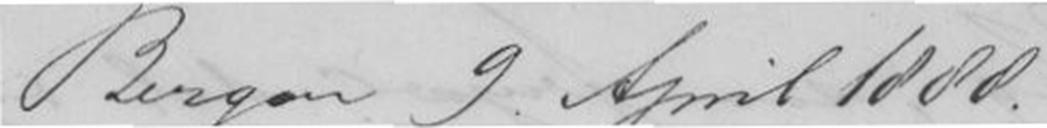Bergen 9. April 1888.
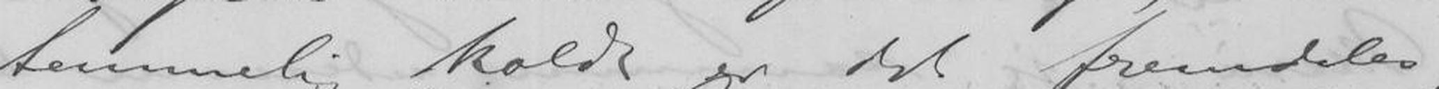temmelig koldt er det fremdeles,
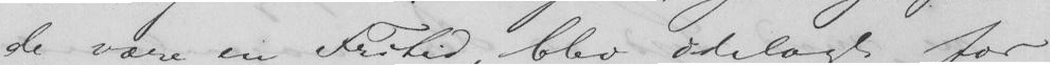de være en Fritid, blev ødelagt for
